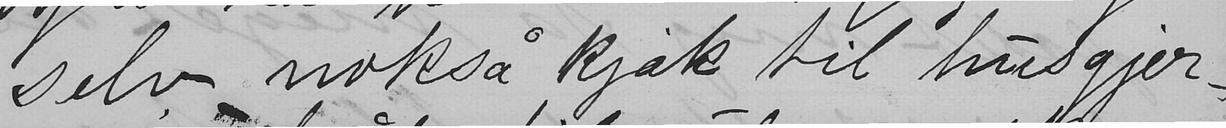selv nokså kjæk til husgjer-
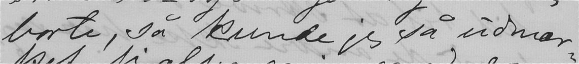borte, så kunde jeg så udmær-
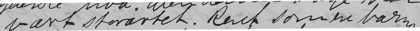vært storartet. Rent som en barm-
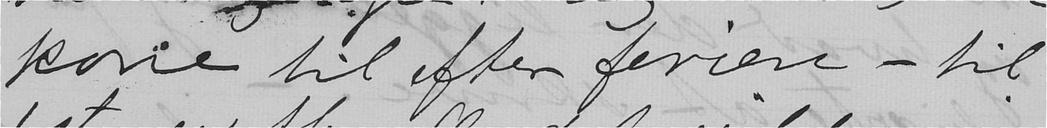kone til efter ferien - til
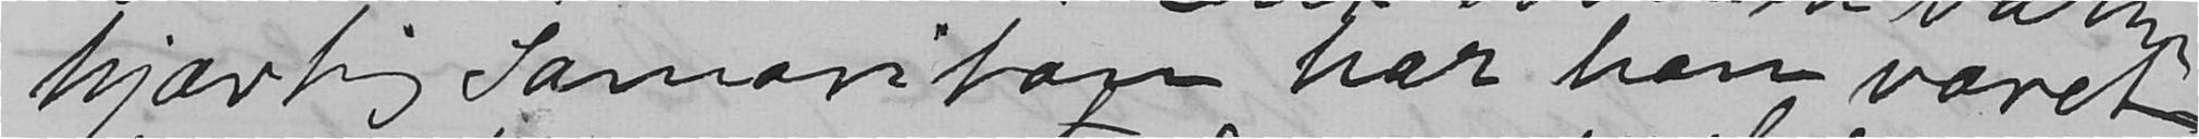hjærtig Samaritan har han været,
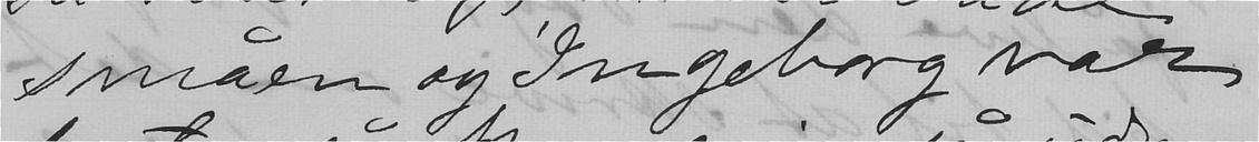småen og Ingeborg var
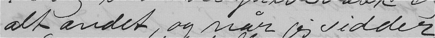alt andet, og når jeg sidder
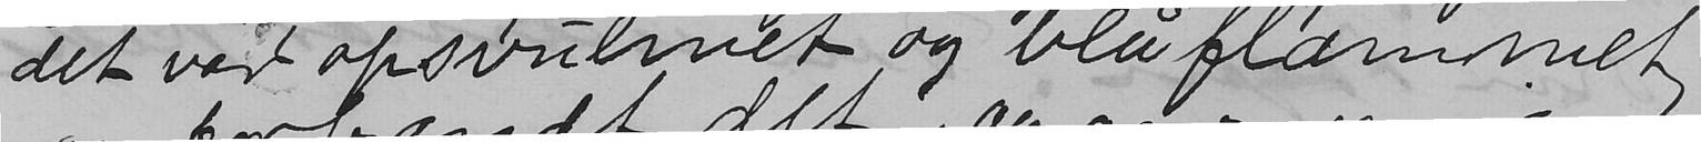det var opsvulmet og blåflammet,
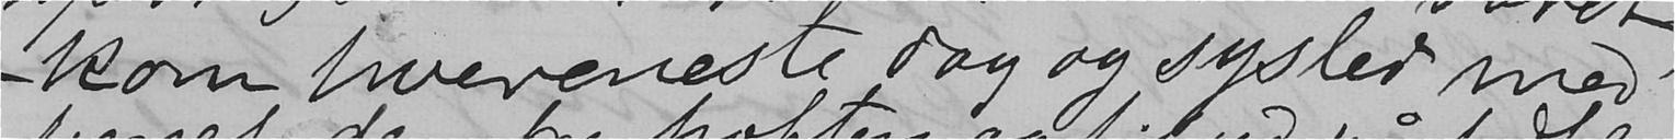- kom hvereneste dag og sysled med
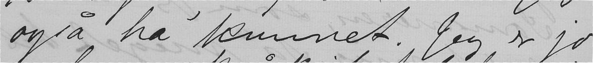også ha' kunnet. Jeg er jo
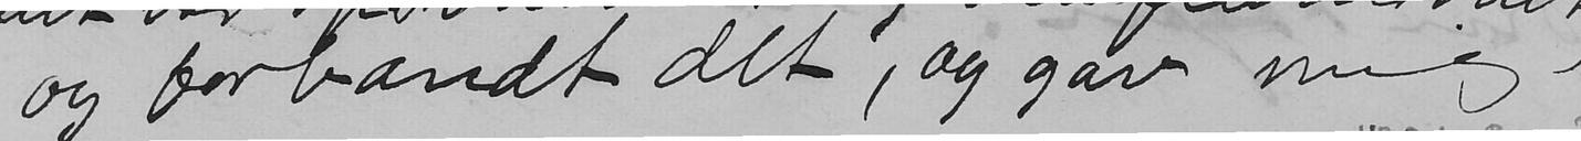og forbandt det, og gav mig
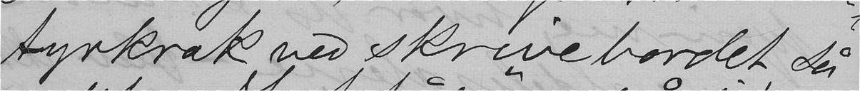tyrkrak ved skrivebordet, så
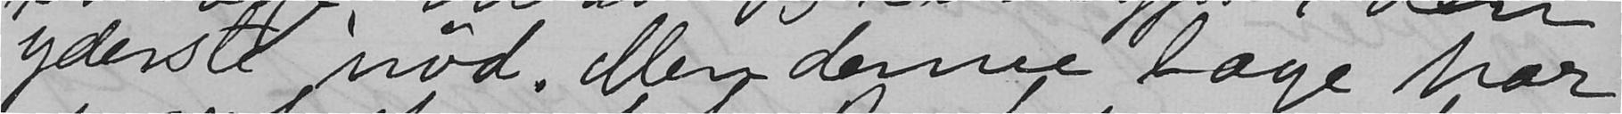yderste nød. Men denne læge har
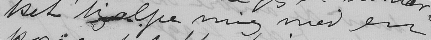ket hælpe mig med en
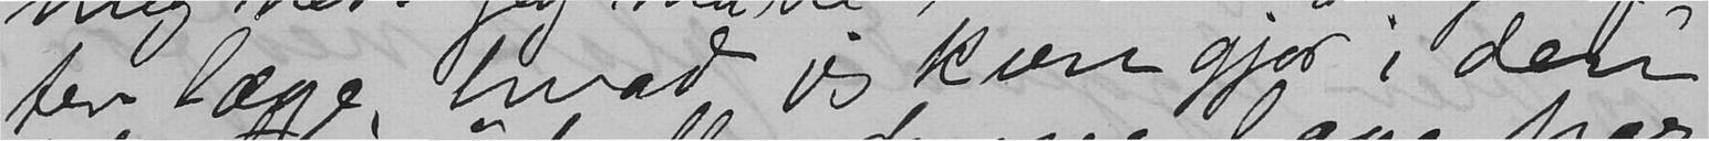ter læge, hvad jeg kun gjør i den
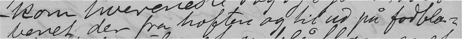benet, der fra hoften og til ud på fodbla-
